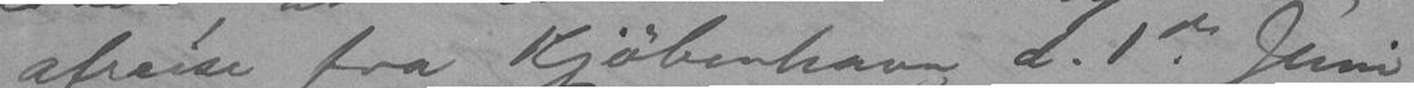afreise fra Kjøbenhavn d. 1ste Juni
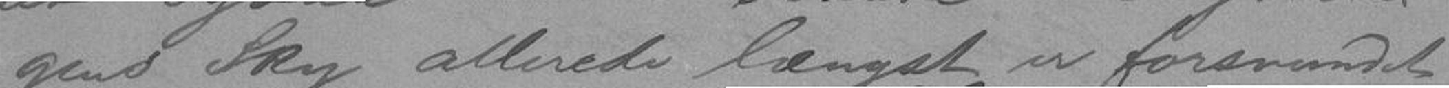gens Sky allerede længst er forsvundet
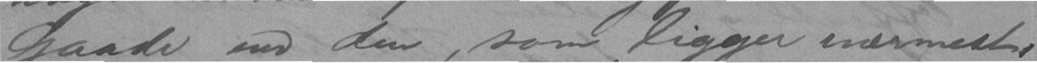Gaade end den, som ligger nærmest.
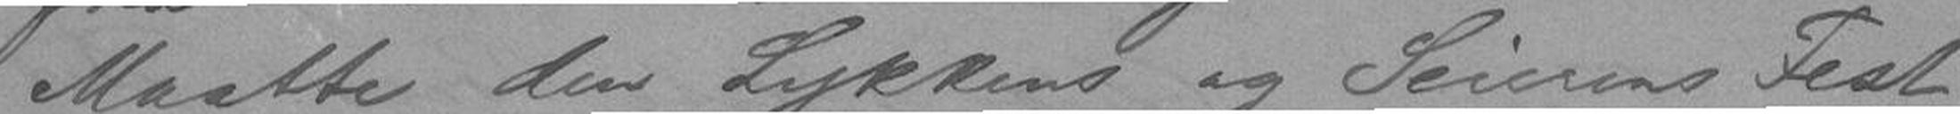Maatte den Lykkens og Seirens Fest,
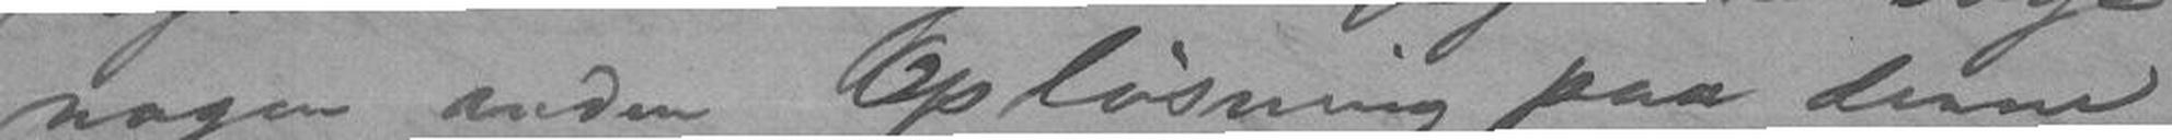nogen anden Opløsning paa denne
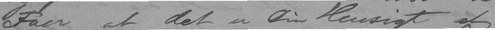Faer, at det er Din Hensigt at
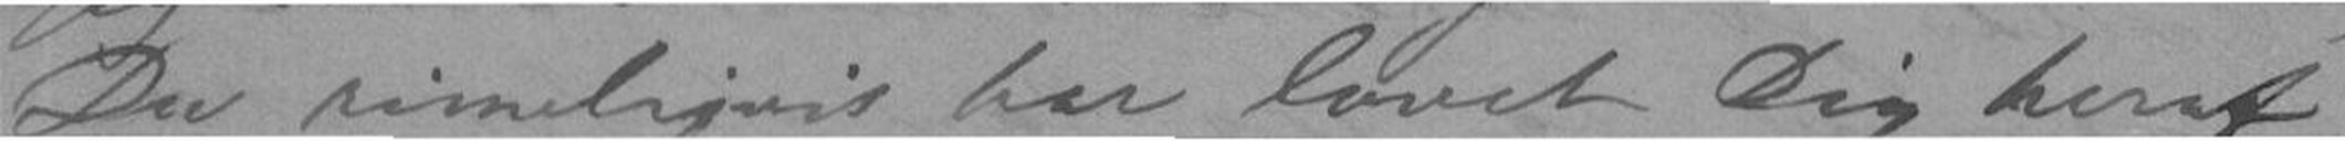Du rimeligvis har lovet Dig heraf,
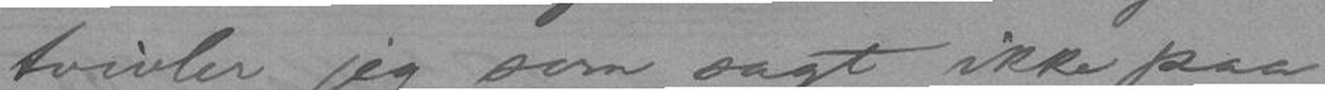tvivler jeg som sagt ikke paa,
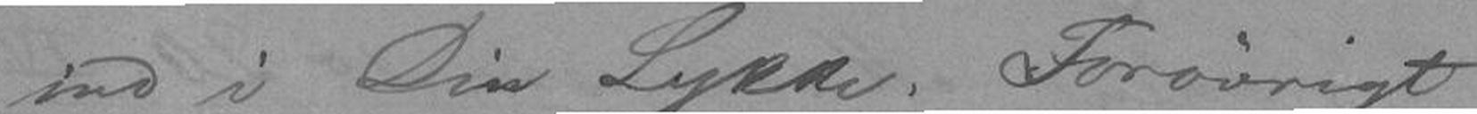ind i Din Lykke. Forøvrigt
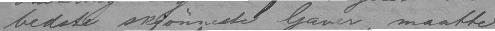bedste, skjønneste Gaver, maatte
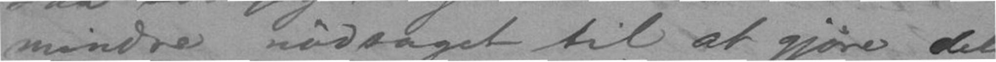mindre nødsaget til at gjøre det
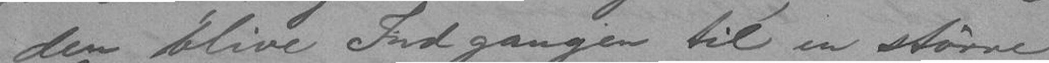den blive Indgangen til en større
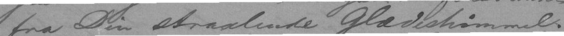fra Din straalende Glædeshimmel.
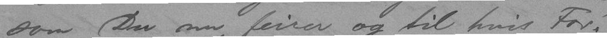som Du nu feirer og til hvis For-
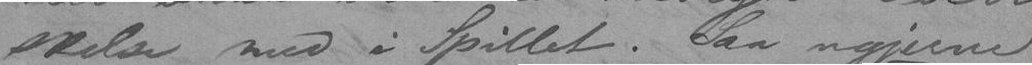skelse med i Spillet. Saa ugjerne
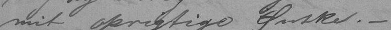mit oprigtige Ønske. -
